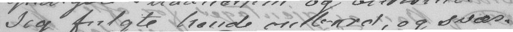Jeg fulgte hende ombord, og svær-
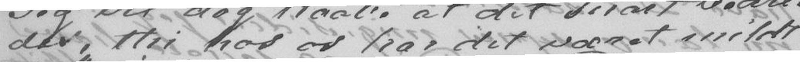des, thi hos os har det været mildt
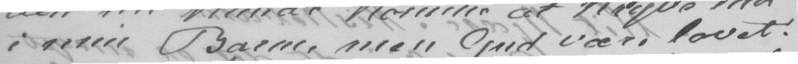i min Barm, men Gud være lovet,
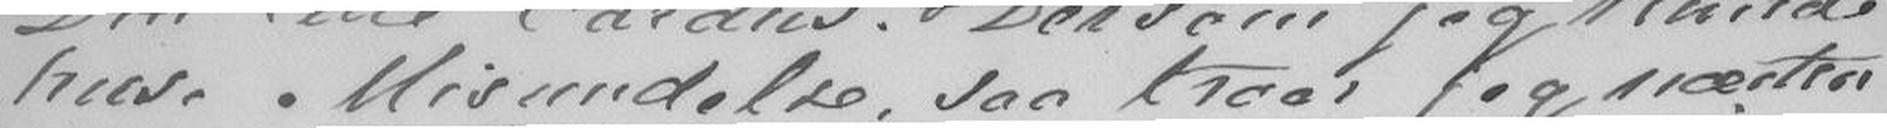have Misundelse saa troer jeg næsten
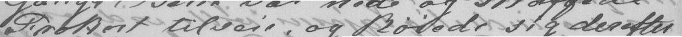Frokost tilveie, og køiede sig derefter
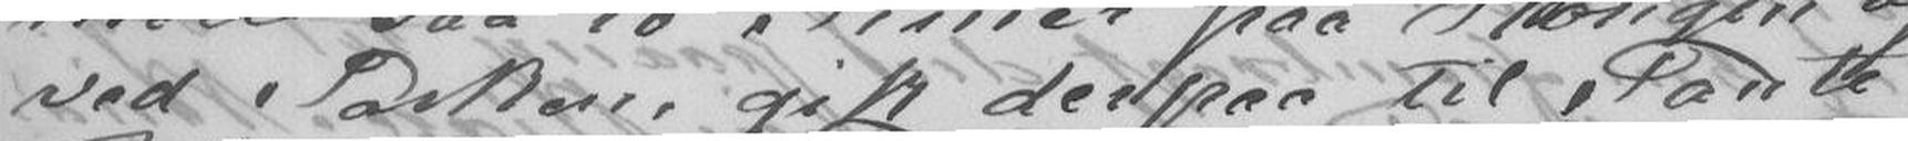ved Parken, Derpaa til Tante
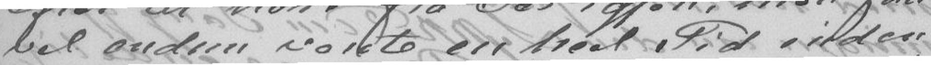vel endnu vente en heel Tid inden
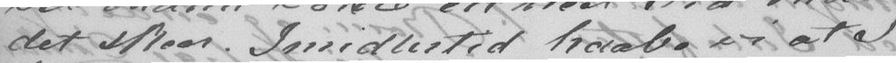det skeer. Imidlertid haabe vi at I
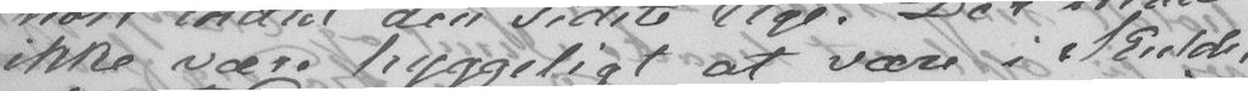ikke være hyggeligt at være i Kulde,
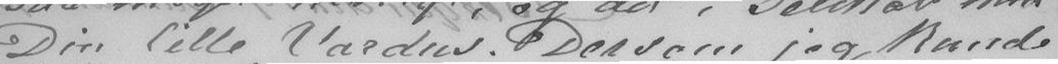Din lille Vardus. Dersom jeg kunde
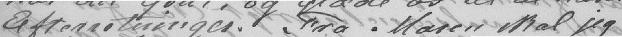Efterretninger. Fra Maren skal jeg
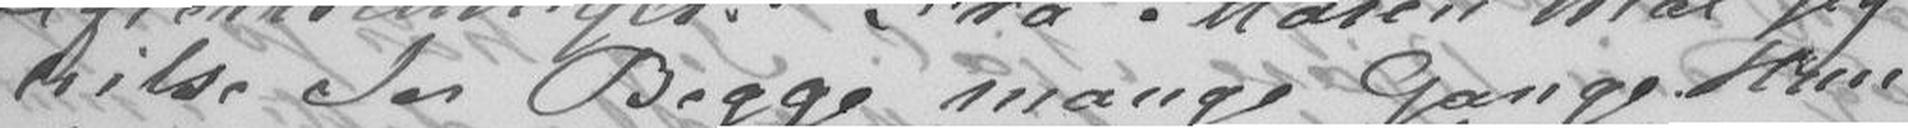hilse Jer Begge mange Gange. Hun
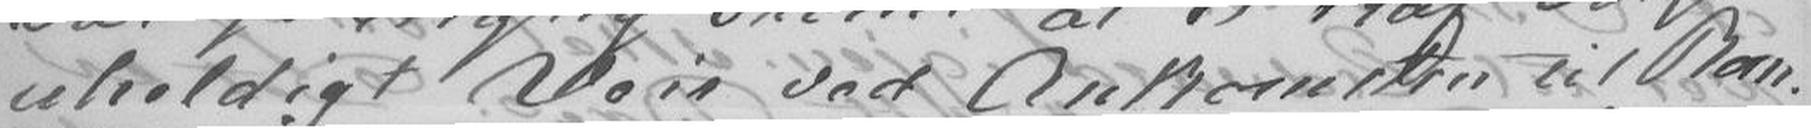uheldigt Veir ved Ankomsten til Rom.
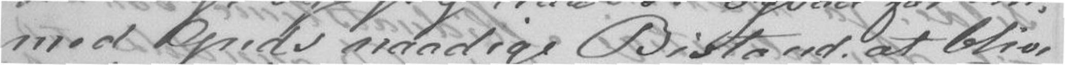med Guds naadige Bistand at blive
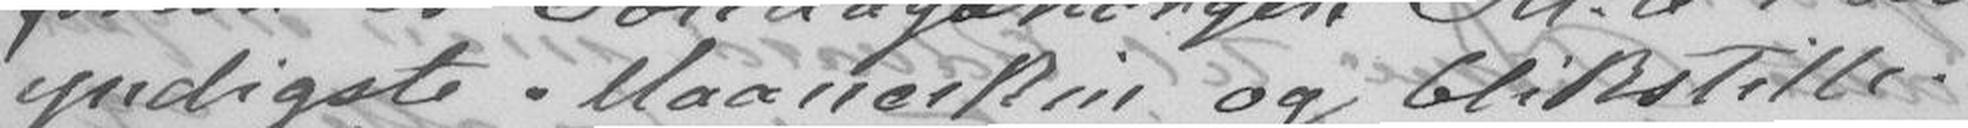yndigste Maaneskin og blikstille.
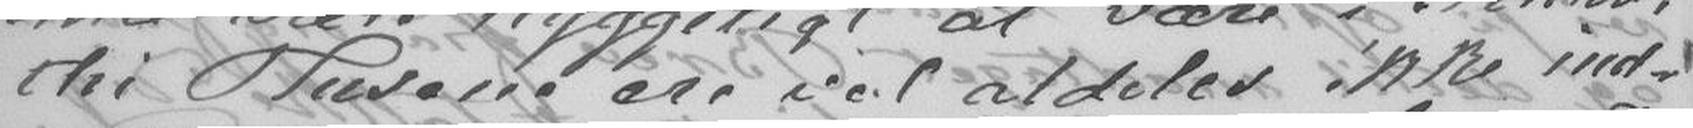thi Husene ere vel aldeles ikke ind-
